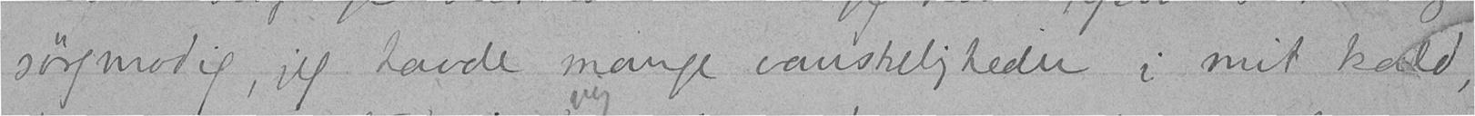sørgmodig, jeg havde mange vanskeligheder i mit kald,
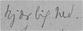kjærlighed.
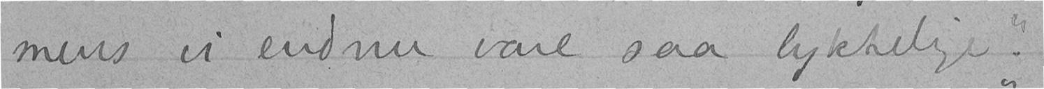mens vi endnu vare saa lykkelige."
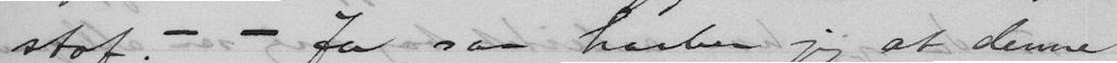stof. - - Ja saa haaber jeg at denne
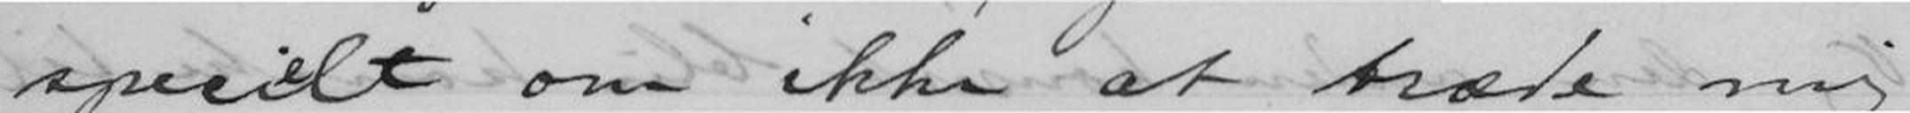specielt om ikke at træde mig
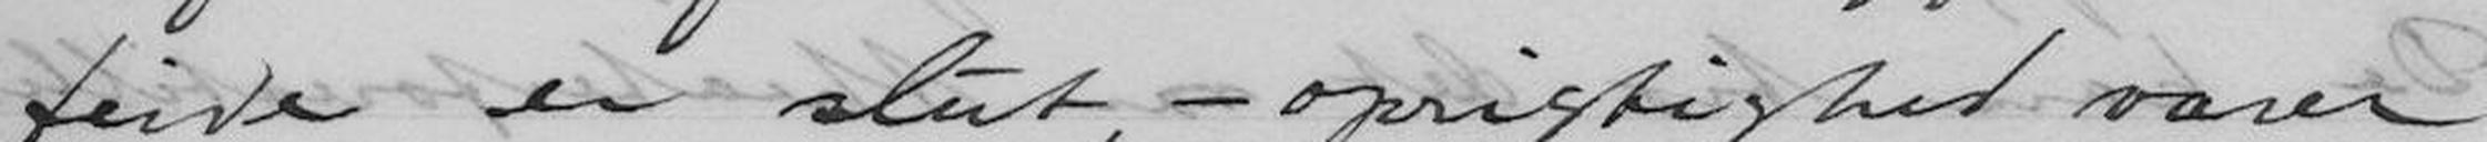feide er slut, - oprigtighed varer
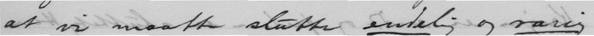at vi maatte slutte endelig og varig
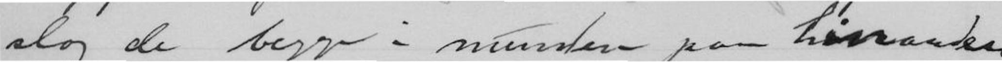slog de begge i munden paa hinanden
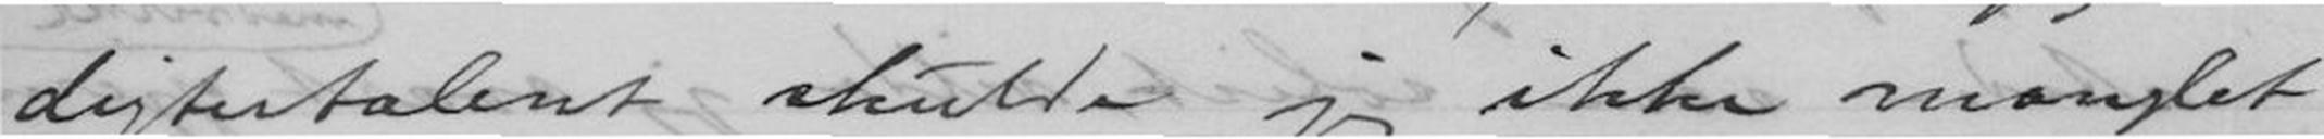digtertalent skulle jeg ikke manglet
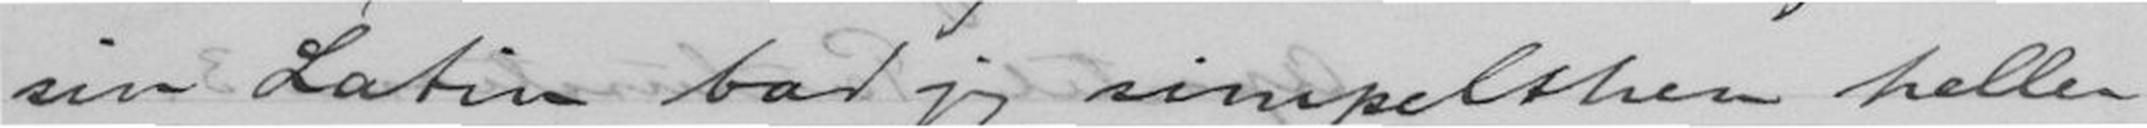sin Latin bad jeg simpelthen heller
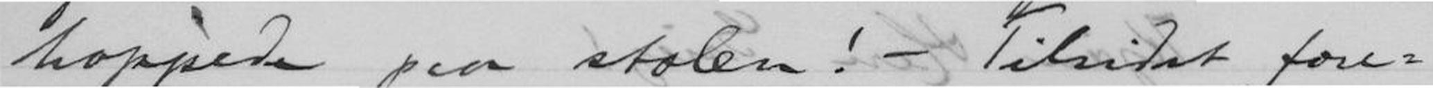hoppede paa stolen! - Tilsidst fore-
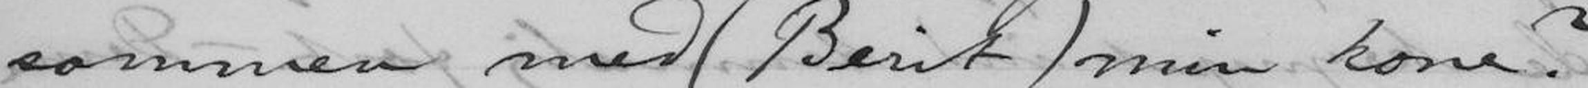sammen med (Berit) min kone?
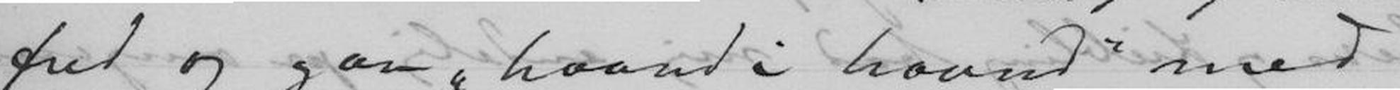fred og gaa "haand i haand" med
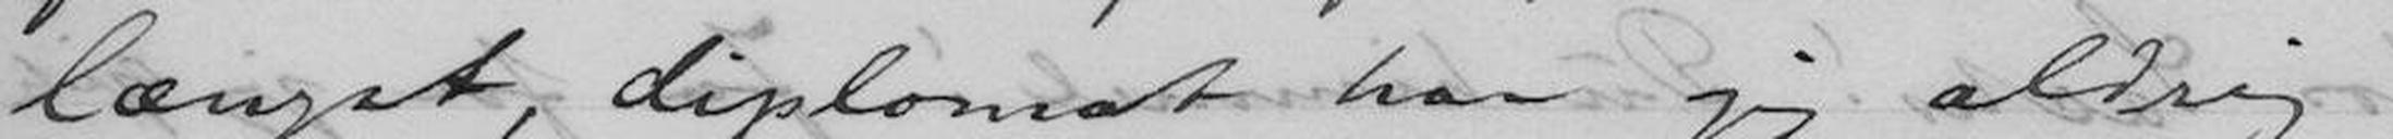længst, diplomat har jeg aldrig
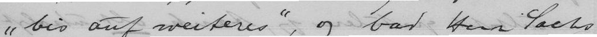"bis auf weiteres", og bad Herr Sachs
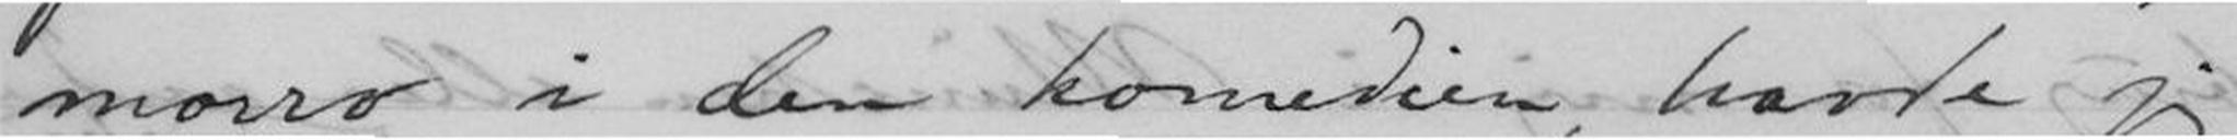morro i den komedien, havde jeg
